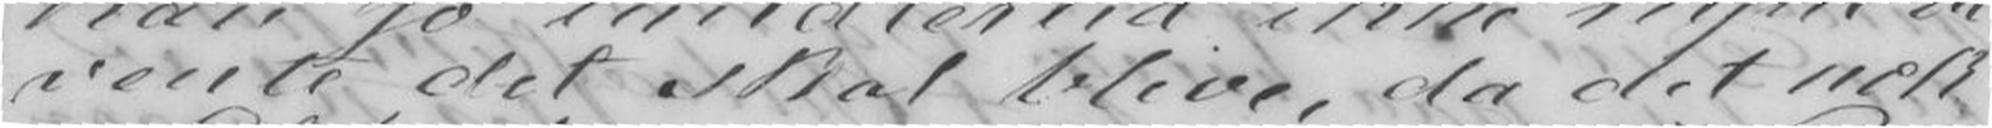vente det skal blive, da det nok
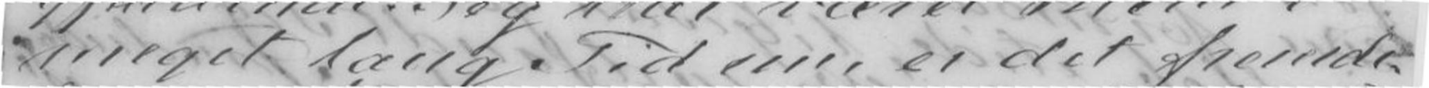meget lang Tid nu, er det fremde-
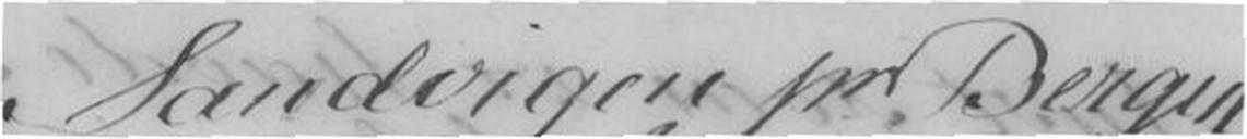Sandvigen pr Bergen
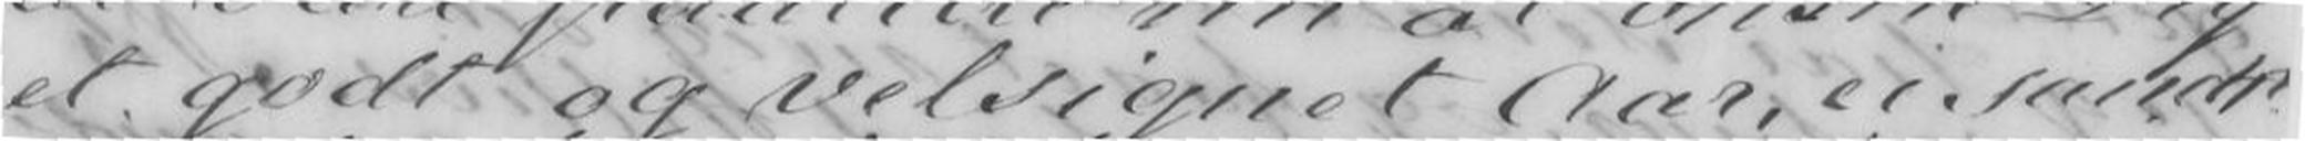et godt og velsignet Aar, ei sandt
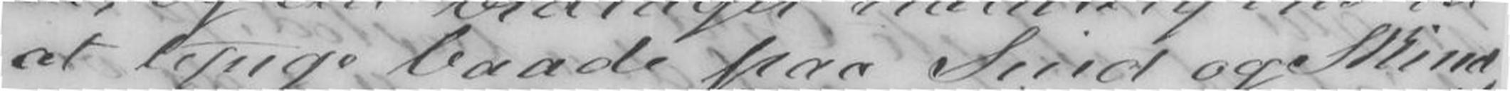at tynge baade paa Sind og Skind,
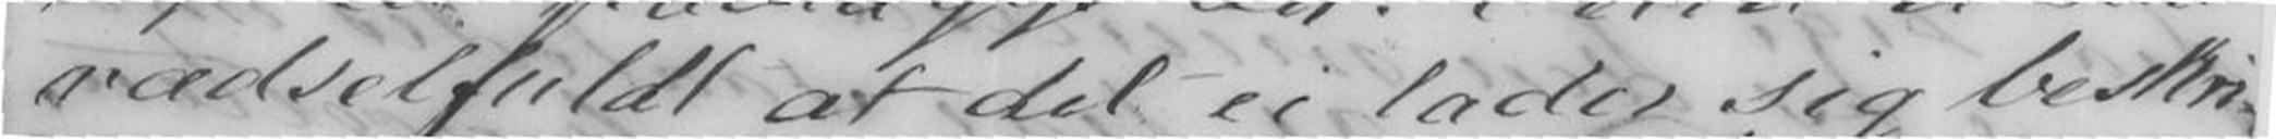rædselfuldt at det ei lade sig beskr-
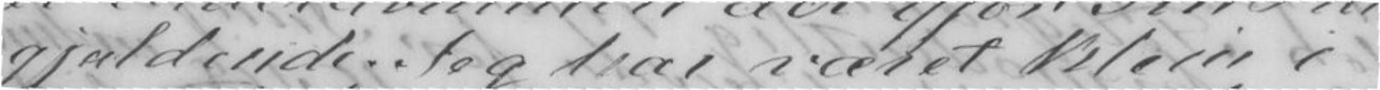gjældende. Jeg har været klein i
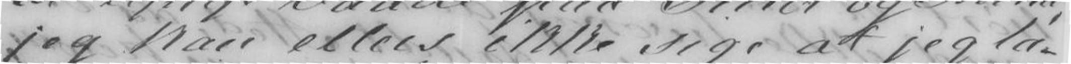jeg kan ellers ikke sige at jeg la-
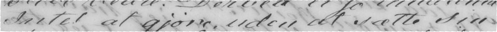intet at gjøre, uden at sætte sin
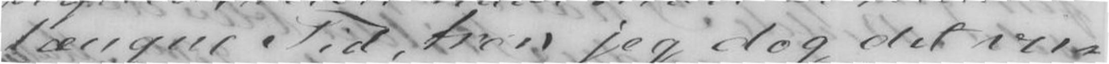længere Tid, trods jeg dog det vir-
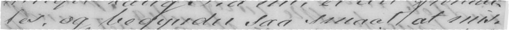les, og begynder saa smaat at mis-
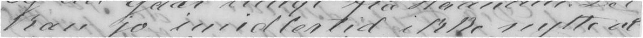kan jo imidlertid ikke nytte at
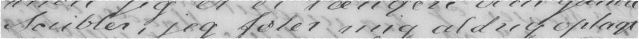Scribler, jeg føler mig aldrig oplagt
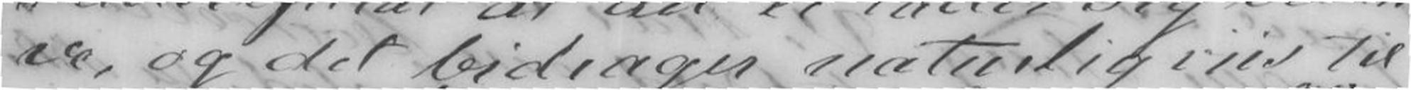ve, og det bidrager naturligviis til
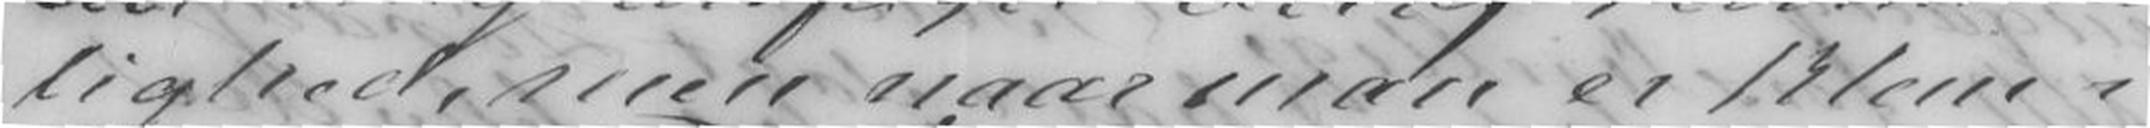lighed, men naar man er klein i
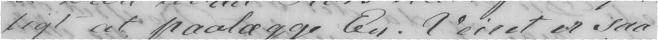ligt at paalægge En. Veiret er saa
